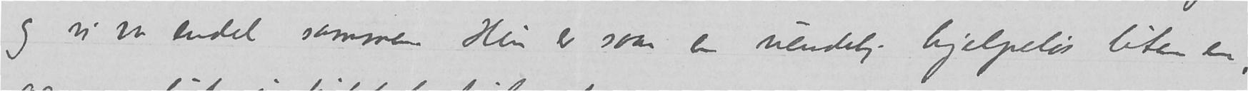og vi var en del sammen. Hun er saan en uendelig hjelpeløs liten en,
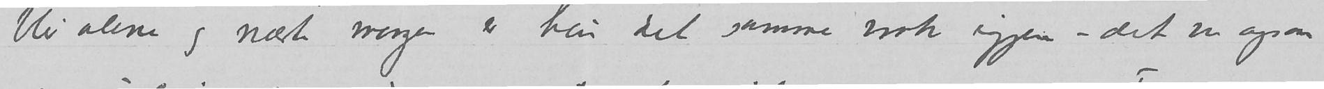blir alene og næste morgen er hun det samme vrak igjen – det var ogsaa
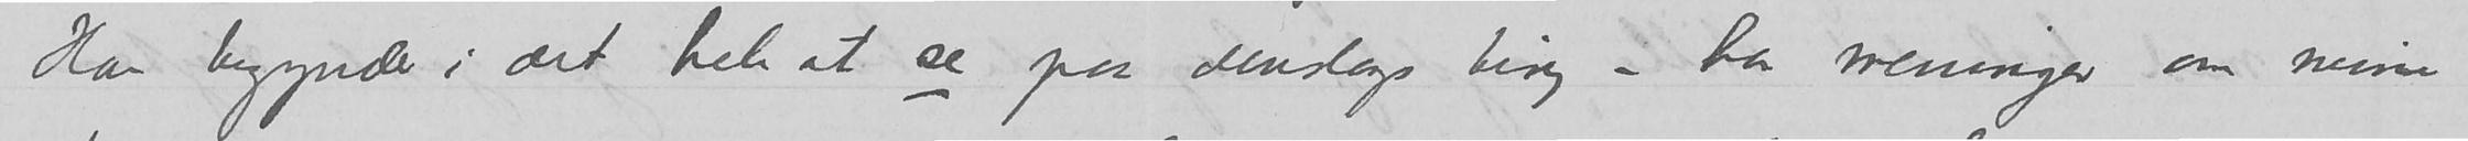Han begynder i det hele at se paa denslags ting – har meninger om mine
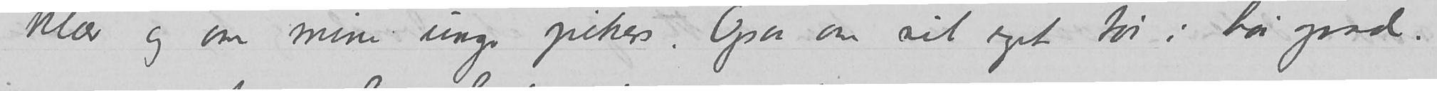klær og om mine unge pikers. Ogsaa om sit eget tøi i høi grad.
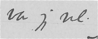var jeg vel.
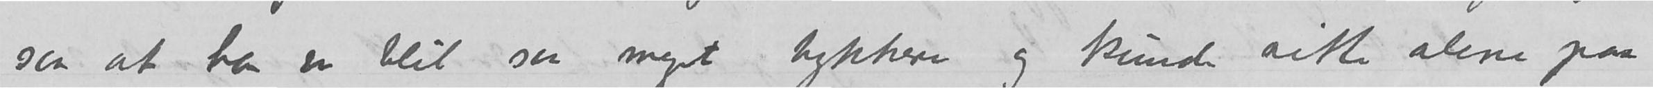saa at han er blit saa meget tykkere og kunde sitte alene paa
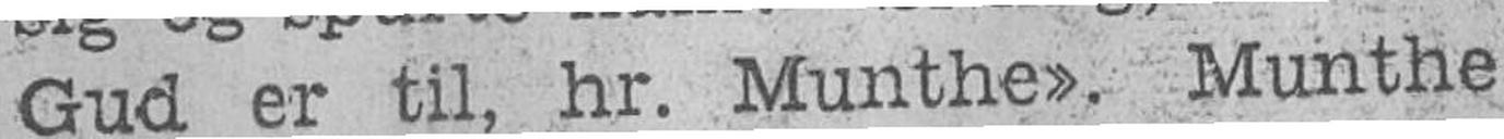Gud er til, hr. Munthe». Munthe
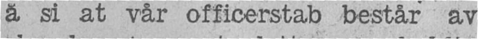å si at vår officerstab består av
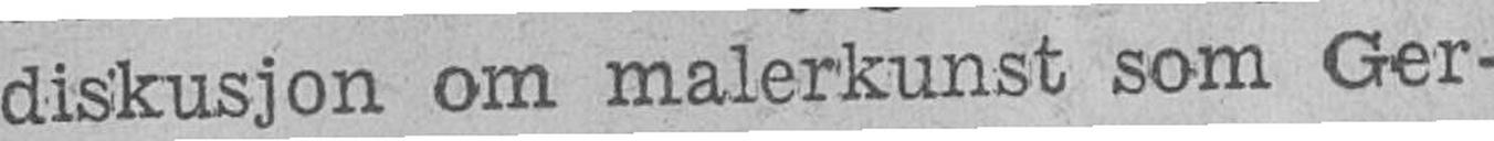diskusjon om malerkunst som Ger-
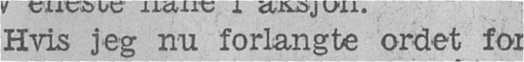Hvis jeg nu forlangte ordet for
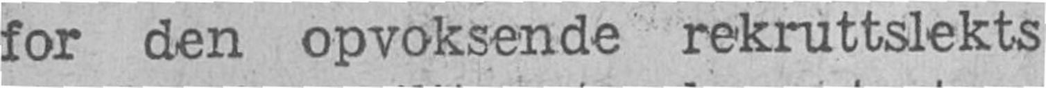for den opvoksende rekruttslekts
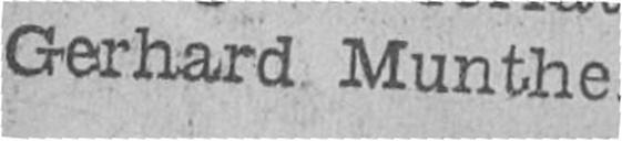Gerhard Munthe
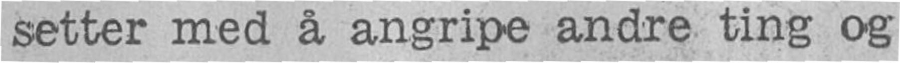setter med å angripe andre ting og
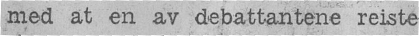med at en av debattantene reiste
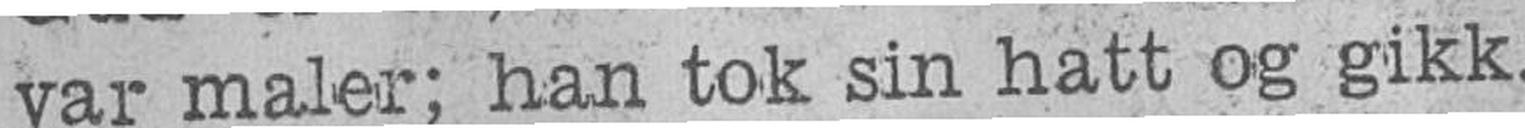var maler; han tok sin hatt og gikk.
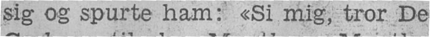sig og spurte ham: «Si mig, tror De
